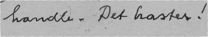handle. Det haster!
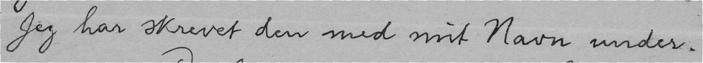Jeg har skrevet den med mit Navn under.
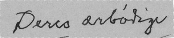Deres ærbødige
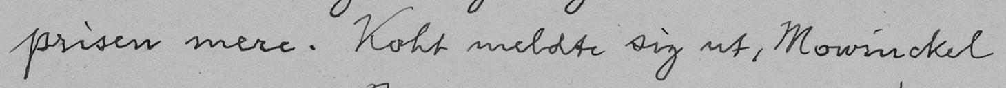prisen mere. Koht meldte sig ut, Mowinckel
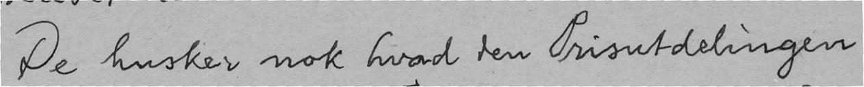De husker nok hvad den Prisutdelingen
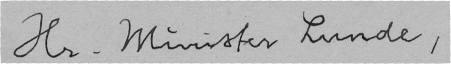Hr. Minister Lunde,
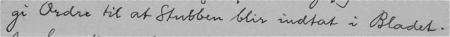gi Ordre til at Stubben blir indtat i Bladet.
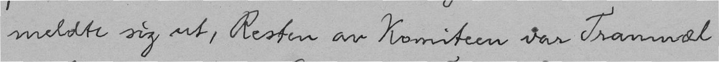meldte sig ut, Resten av Komiteen var Tranmæl
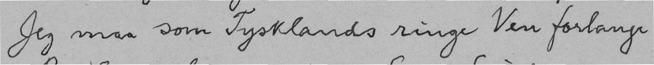Jeg maa som Tysklands ringe Ven forlange
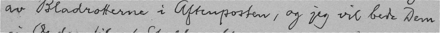av Bladrotterne i Aftenposten, og jeg vil bede Dem
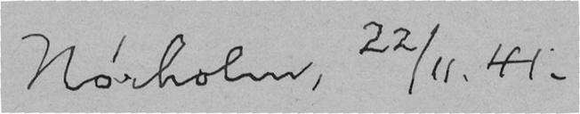Nørholm, 22/11. 41.
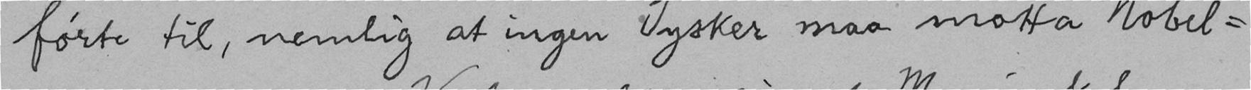førte til, nemlig at ingen Tysker maa motta Nobel-
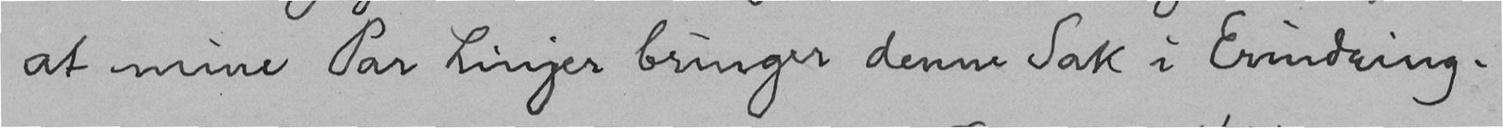at mine Par Linjer bringer denne Sak i Erindring.
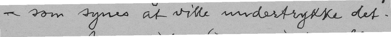- som synes at ville undertrykke det.
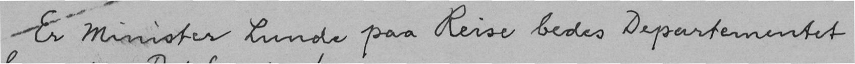Er Minister Lunde paa Reise bedes Departementet
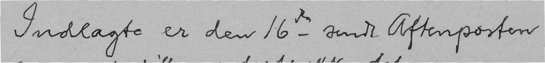Indlagte er den 16de sendt Aftenposten
